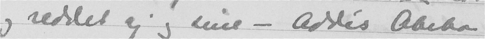og reddet sig og sine - Addis Abeba
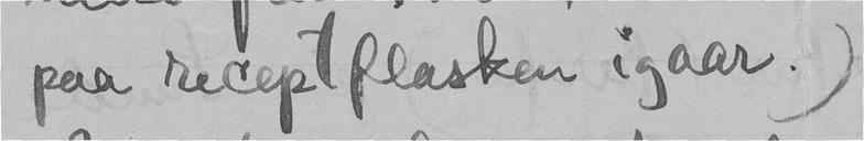paa receptflasken igaar.)
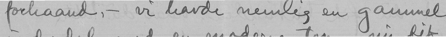forhaand, - vi havde nemlig en gammel
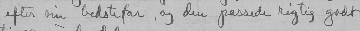efter sin bedstefar, og den passede rigtig godt
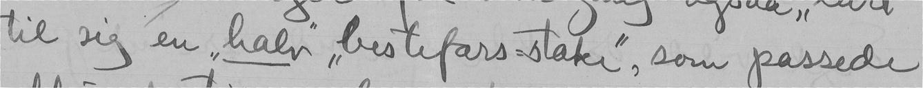til sig en "halv" "bestefars-stake", som passede
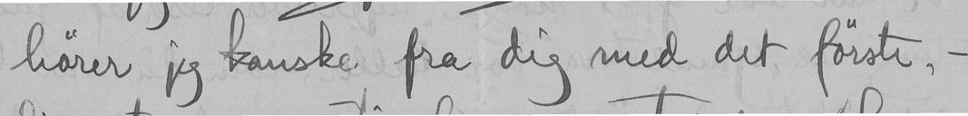hører jeg kanske fra dig med det første, -
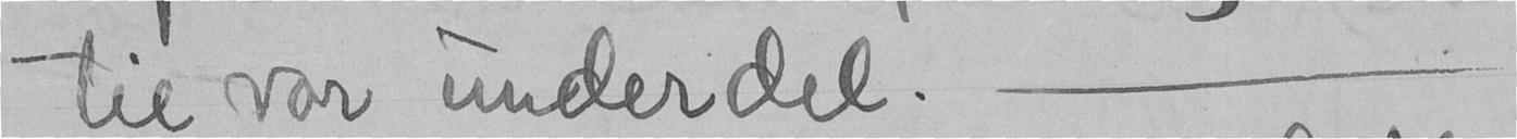til vor underdel. -
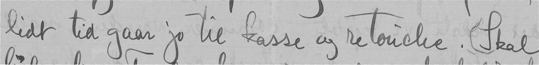lidt tid gaar jo til kasse og retouche. Skal
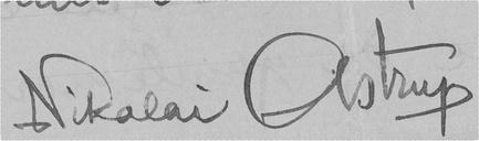Nikolai Astrup
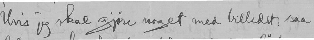Hvis jeg skal gjøre noget med billedet, saa
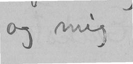og mig
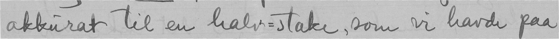akkurat til en halv-stake, som vi havde paa
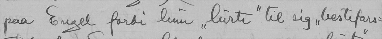paa Engel fordi hun "lurte" til sig "bestefars-
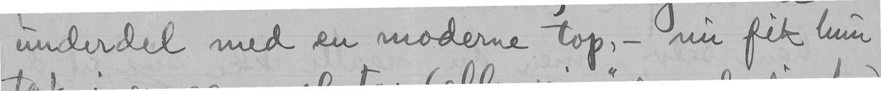underdel med en moderne top, - nu fik hun
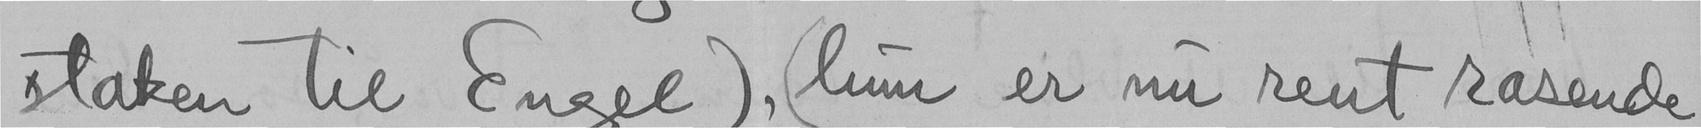staken til Engel), (hun er nu rent rasende
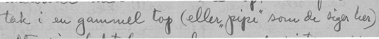tak i en gammel top (eller "pipe" som de siger her)
